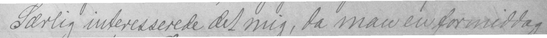Særlig interesserede det mig, da man en formiddag
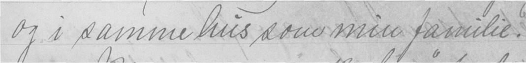og i samme hus som min familie.
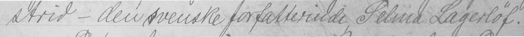strid - den svenske forfatterinde, Selma Lagerløf.
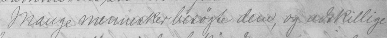Mange mennesker besøgte dem, og adskillige
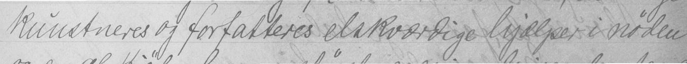kunstneres og forfatteres elskværdige hjælper i nøden
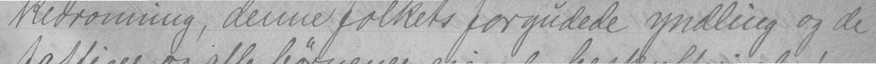kedronning, denne folkets forgudede yndling og de
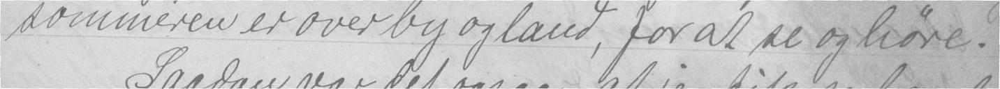sommeren er over by og land, for at se og høre.
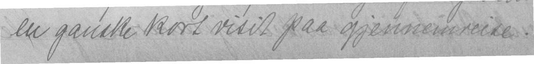en ganske kort visit paa gjennemreise.
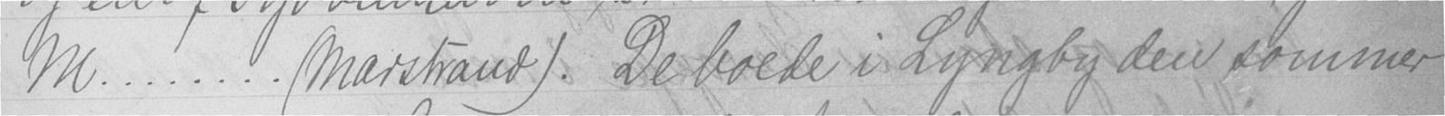M........ (Marstrand). De boede i Lyngby den sommer
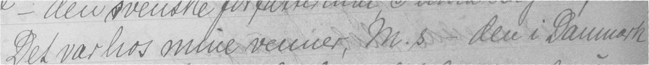Det var hos mine venner, M.S - den i Danmerk
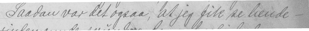Saadan var det ogsaa, at jeg fik se hende -
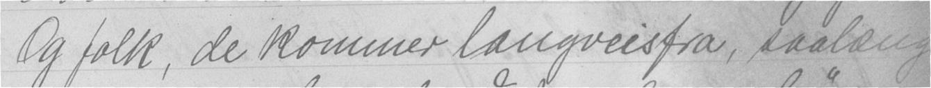Og folk, de kommer langveisfra, saalæng
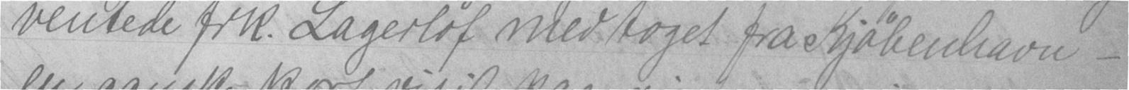ventede frk. Lagerløf med toget fra Kjøbenhavn -
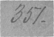351.
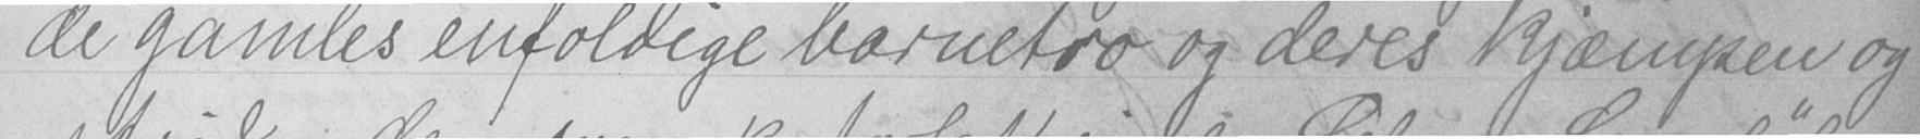de gamles enfoldige barnetro og deres kjæmpen og
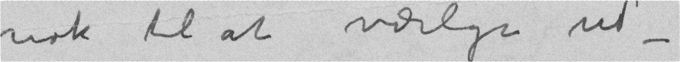nok til at vælge ud –
